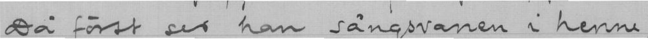Då först ser han sångsvanen i henne,
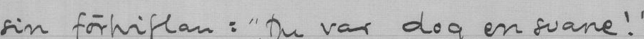sin förtviflan: "Du var dog en svane!"
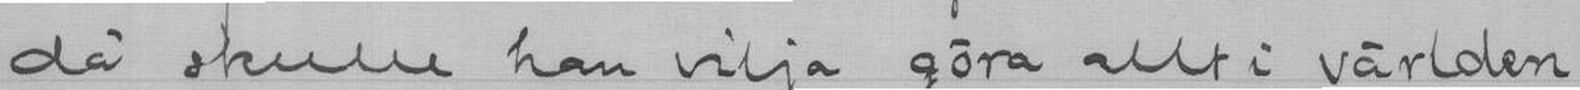då skulle han vilja göra allt i världen
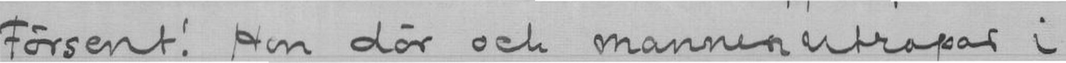Försent! Hon dör och mannen utropar i
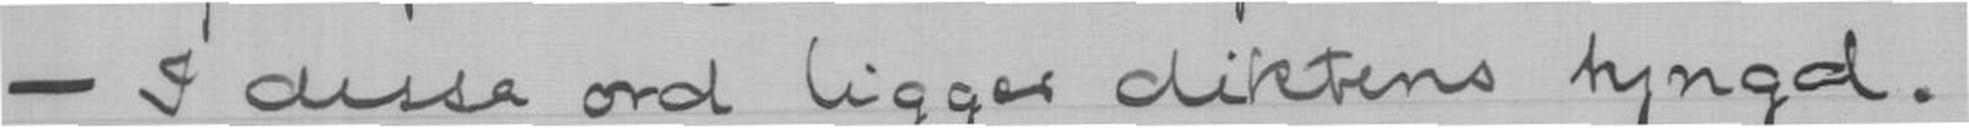- I dessa ord ligger diktens tyngd.
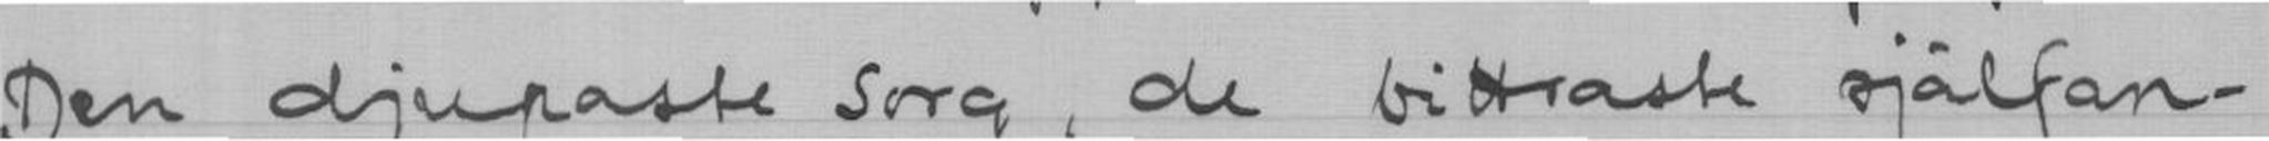Den djupaste sorg, de bittraste själfan-
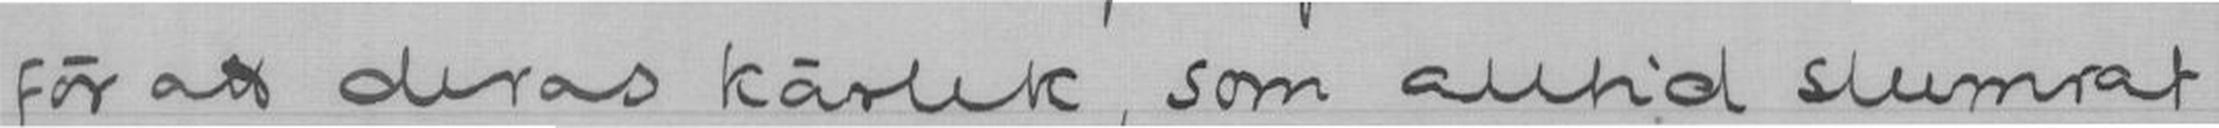för att deras kärlek, som alltid slumrat
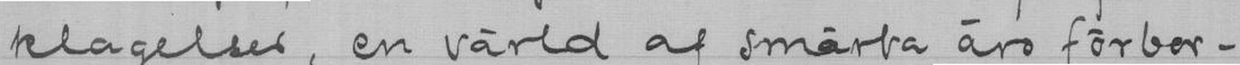klagelser, en värld af smärta äro förbor-
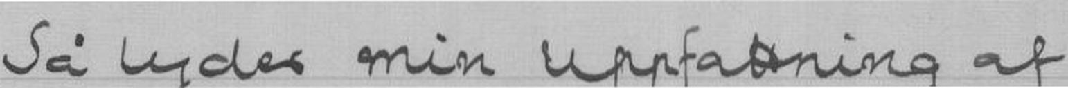Så lyder min uppfattning af
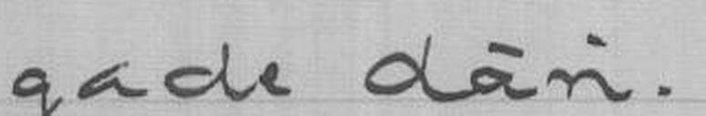gade däri.
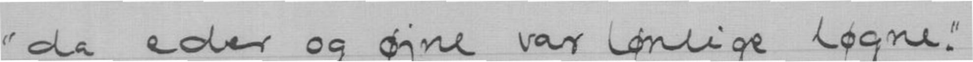"da eder og øjne var lønlige løgne".
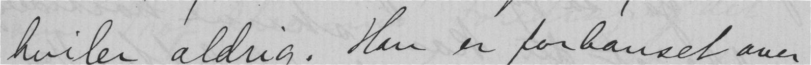hviler aldrig. Han er forbauset over
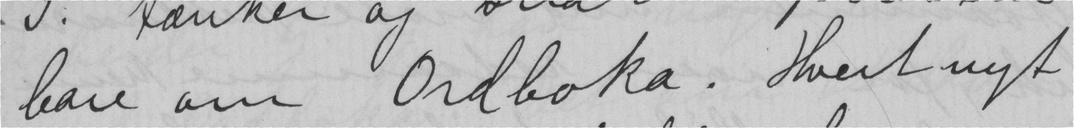bare om Ordboka. Hvert nyt
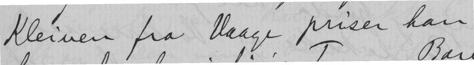Kleiven fra Vaage priser han
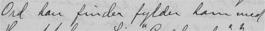Ord han finder fylder ham med
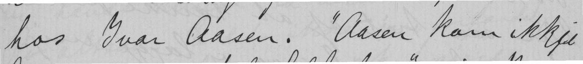hos Ivar Aasen. "Aasen kom ikkje
